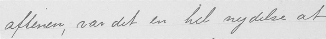aftenen, var det en hel nydelse at
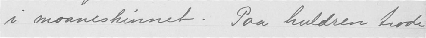i maaneskinnet. Paa huldren trode
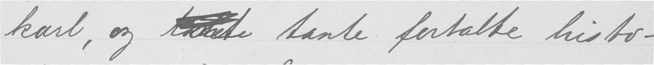karl, og tante tante fortalte histo-
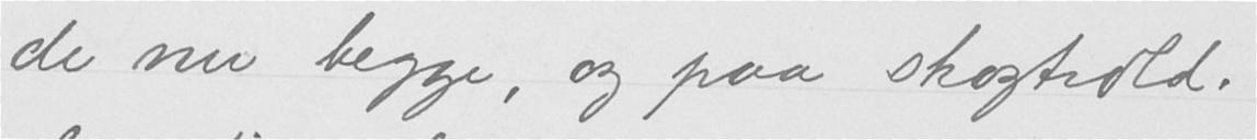de nu begge, og paa skogtrold.
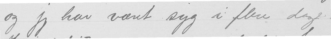og jeg har været syg i flere dage.
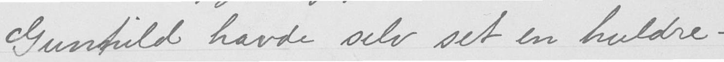Gunhild havde selv set en huldre-
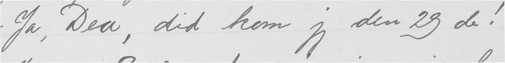- Ja, Dea, did kom jeg den 29 de!
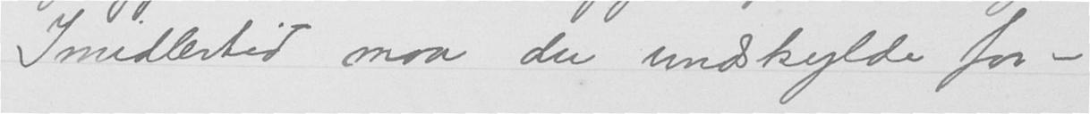Imidlertid maa du undskylde for-
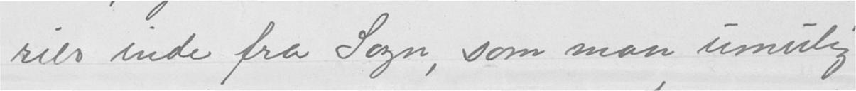rier inde fra Sogn, som man umulig
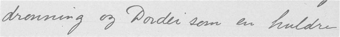dronning og Dordei som en huldre
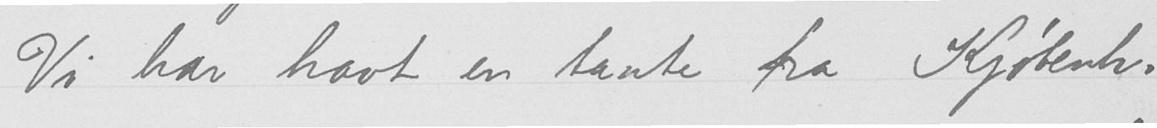Vi har havt en tante fra Kjøbenh.
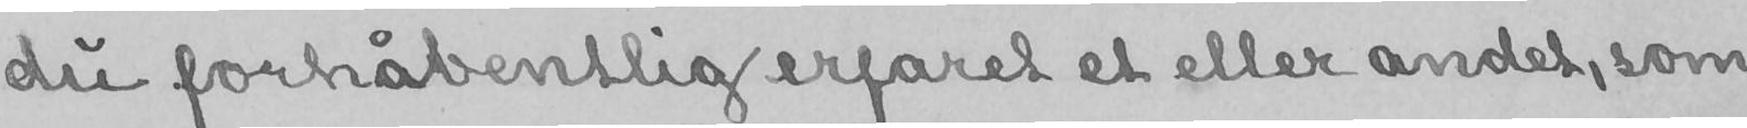du forhåbentlig erfaret et eller andet, som
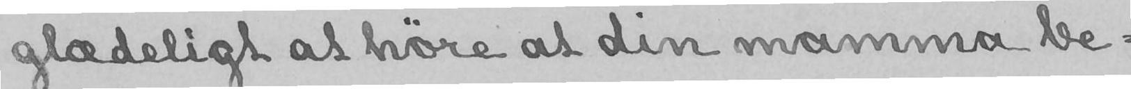glædeligt at høre at din mamma be-
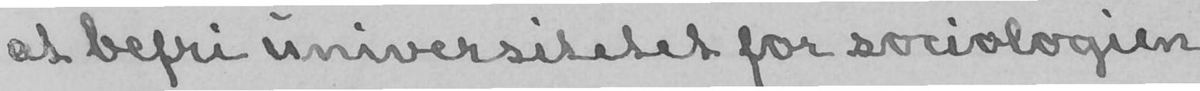at befri universitetet for sociologien
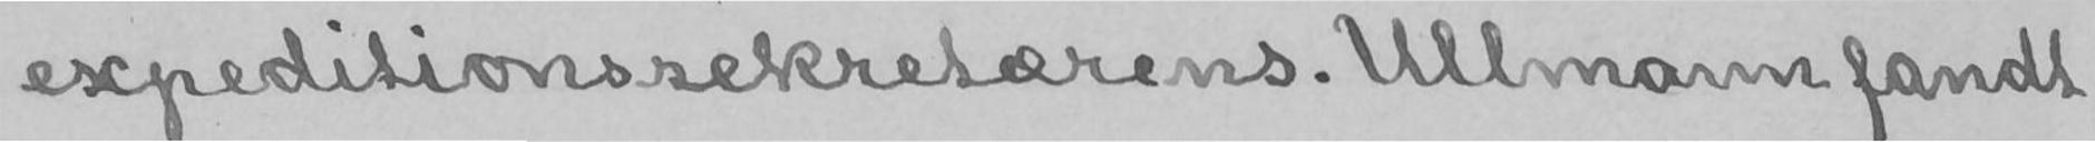expeditionssekretærens. Ullmann fandt
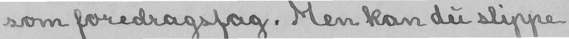som foredragsfag. Men kan du slippe
